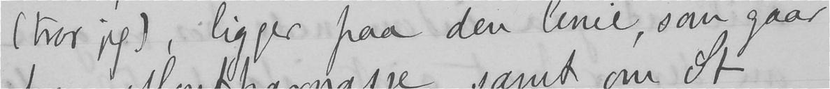(tror jeg), ligger paa den linie, som gaar
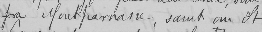fra Montparnasse, samt om St
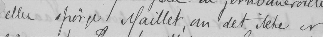eller spørge Maillet, om det ikke er
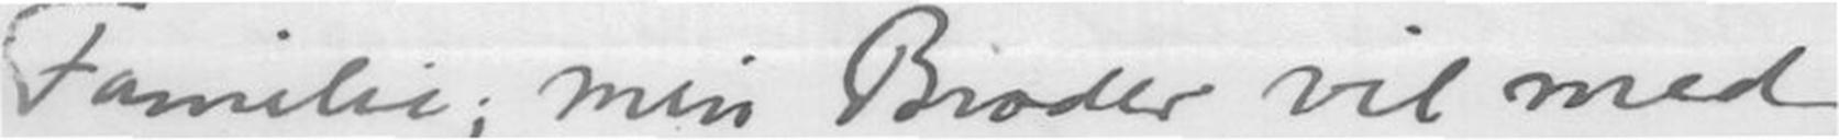Familie, min Broder vil med
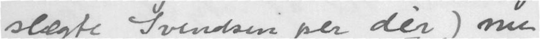slægte Svendsen per dér) nu
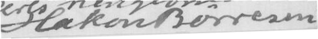Håkon Børresen
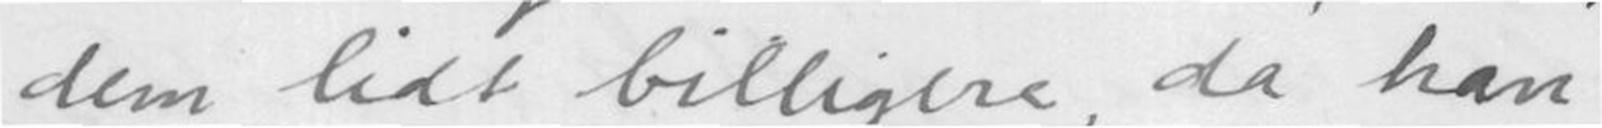dem lidt billigere, da han
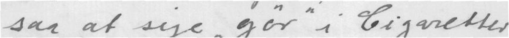saa at sige gør i Cigaretter
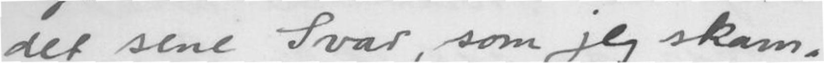det sene Svar, som jeg skam-
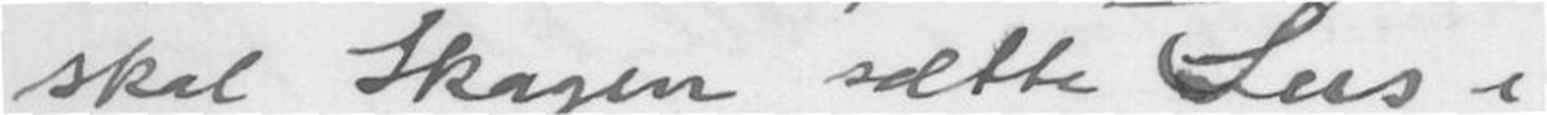skal Skagen sætte Sus i
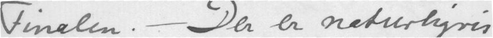Finalen. - Der er naturligvis
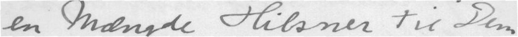en Mængde Hilsner til Dem
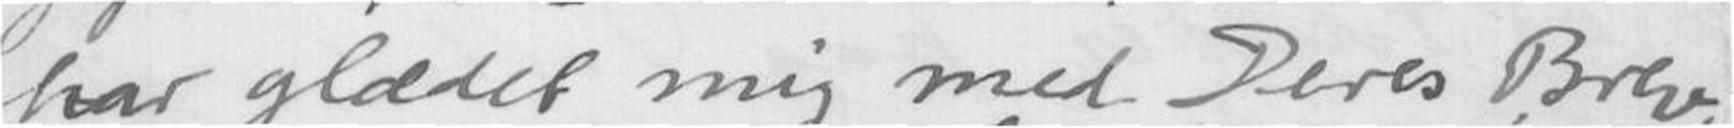har glædet mig med Deres Brev.
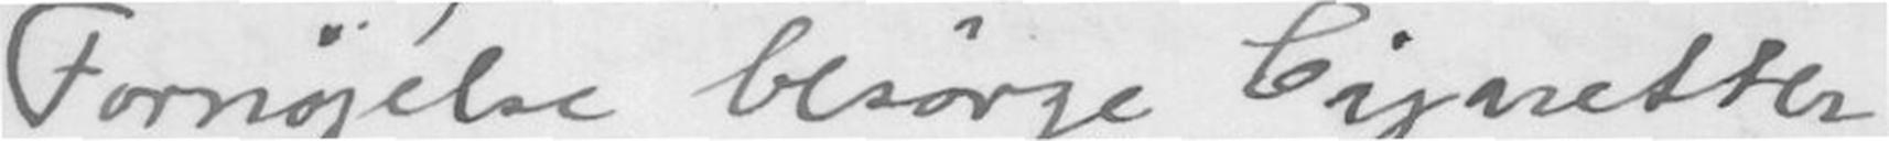Fornøjelse besørge Cigaretter
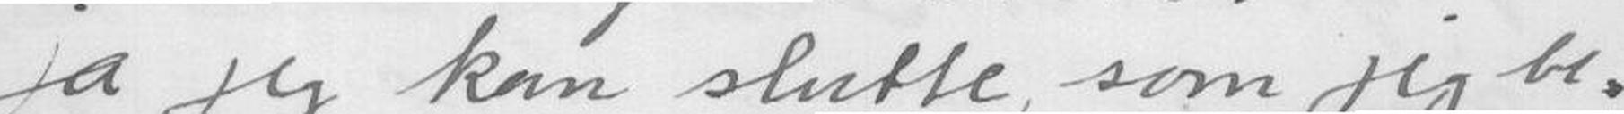Ja jeg kan slutte som jeg be-
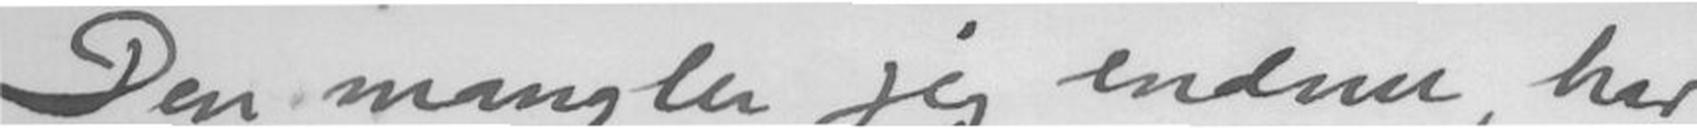Den mangler jeg endnu, har
